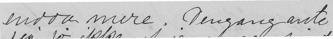endda mere. Dengang ante
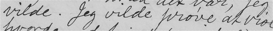vilde. Jeg vilde prøve at vise
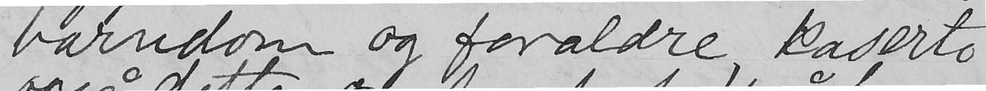barndom og forældre, kaserte
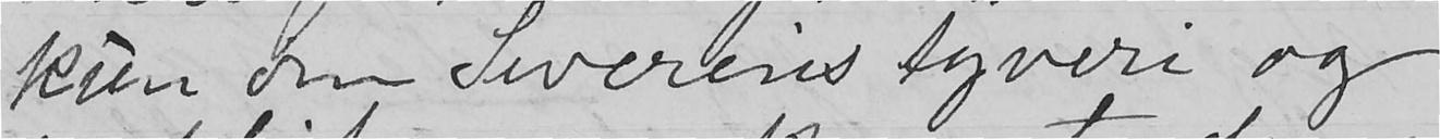kun om Severins tyveri og
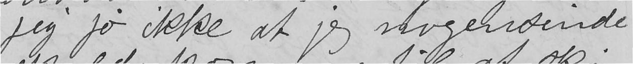jeg jo ikke at jeg nogensinde
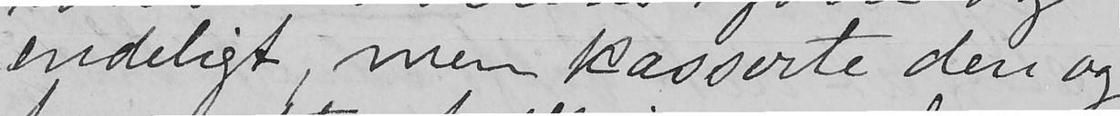endeligt, men kasserte den og
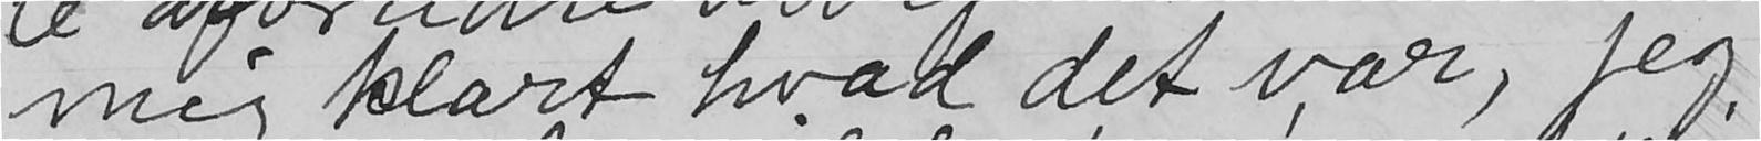mig klart hvad det var, jeg
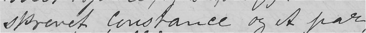skrevet Constance og et par
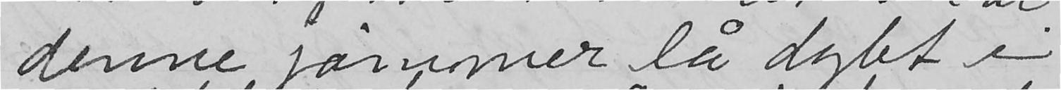denne jammer lå dybt i
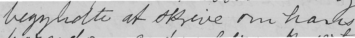begyndte at skrive om hans
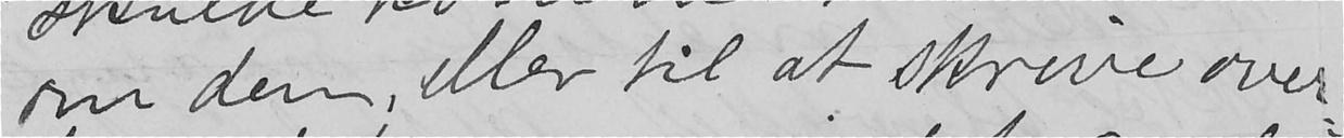om dem, eller til at skrive over-
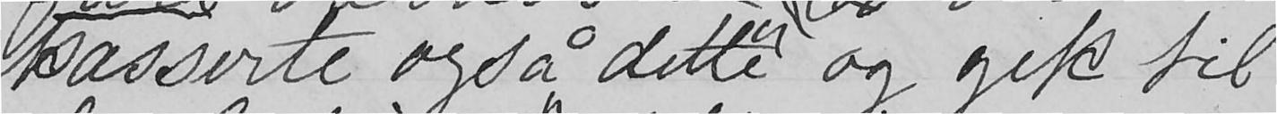kasserte også dette og gik til
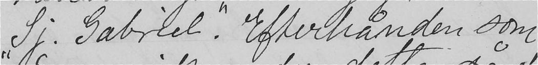"Sj. Gabriel". Efterhånden som
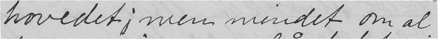hovedet; men mindet om al
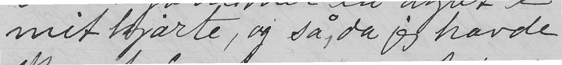mit hjærte, og så, da jeg havde
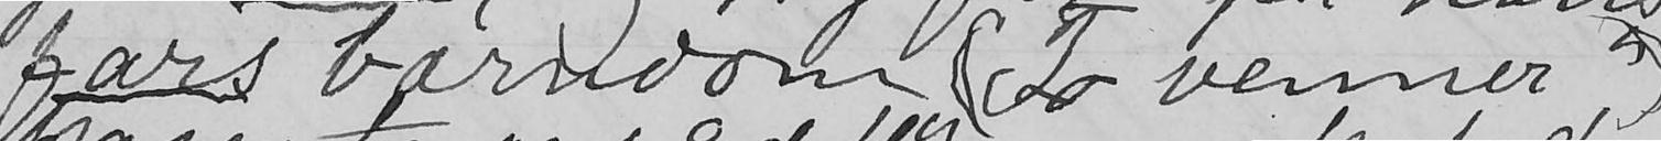fars barndom ("To venner",)
